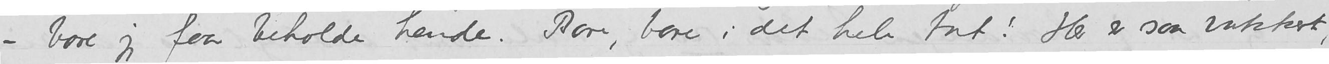-bare jeg faar beholde hende. Bare, bare i det hele tat! Her er saa vakkert,
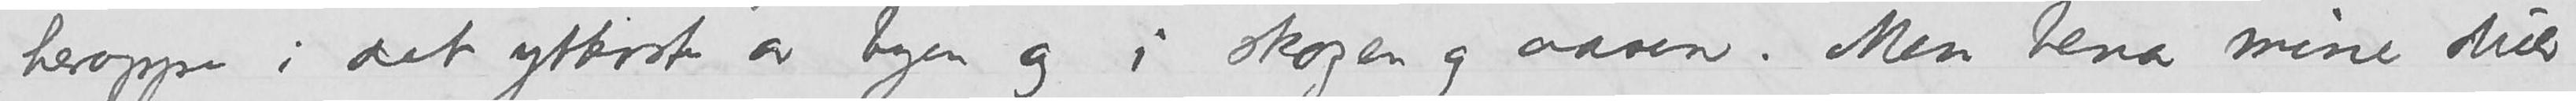heroppe i det ytterste av byen og i skogen og aasen. Men bena mine duer
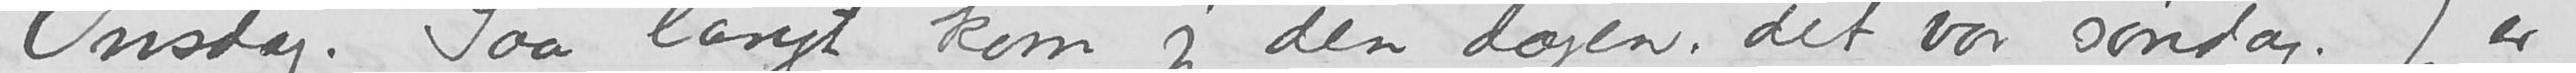OnsdagSaa langt kom jeg den dagen; det var søndag. Jeg er
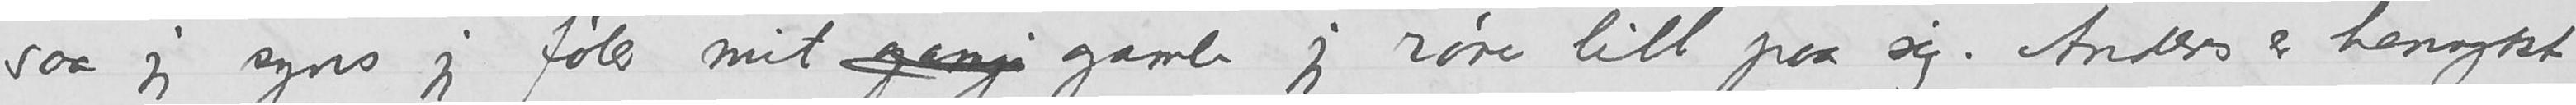saa jeg syns jeg føler mit gange gamle jeg røre litt paa sig. Anders er henrykt
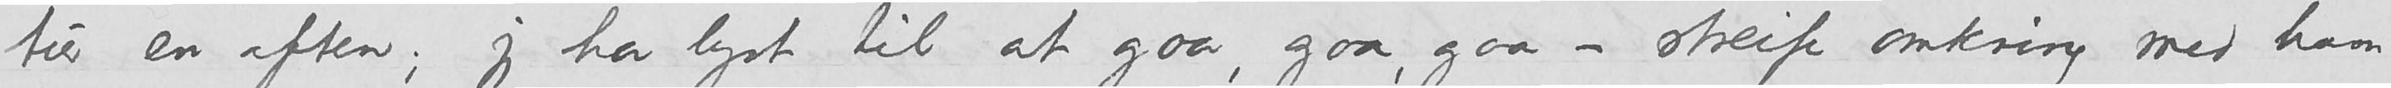tur en aften; jeg har lyst til at gaa, gaa, gaa - streife omkring med ham
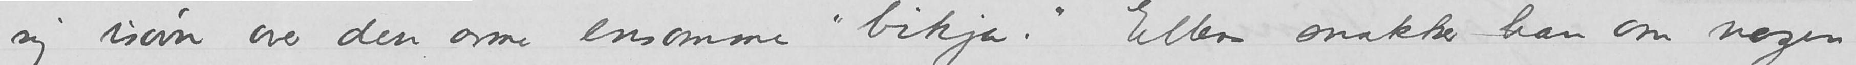sig isøvn over den arme ensomme «bikja.» Ellers snakker han om nogen
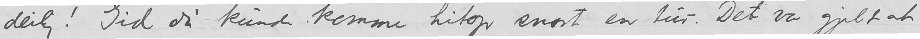deilig! Gid du kunde komme hitop snart en tur. Det var gjilt at
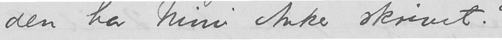den har Nini Anker skrivet.»
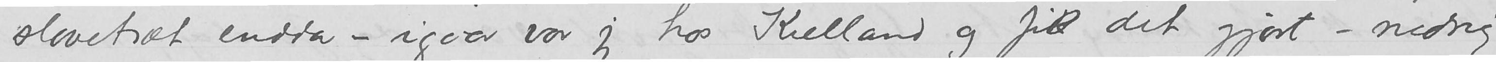slavetræt endda - igaar var jeg hos Kielland og fik det gjort - nedrig
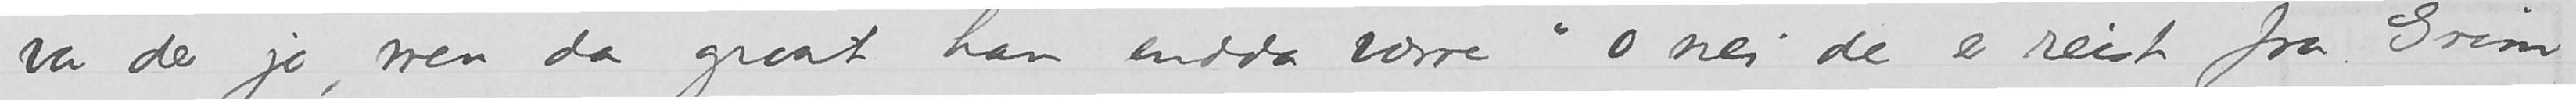var der jo, men da graat han endda værre «o nei de er reist fra Grim
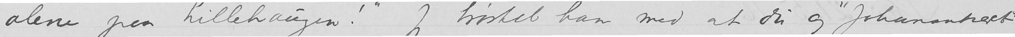alene paa Lillehaugen!» Jeg trøstet ham med at du og «Johansetreet»
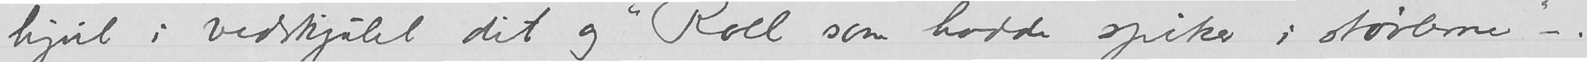hjul i vedskjulet dit og «Roll som hadde spiker i støvlerne» -.
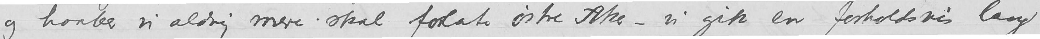og haaber vi aldrig mere skal forlate Østre Aker - vi gik en forholdsvis lang
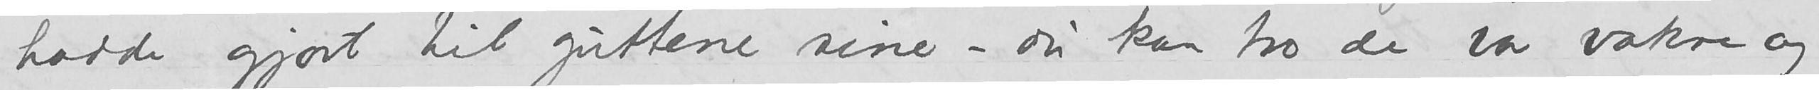hadde gjort til guttene sine – du kan tro de var vakre
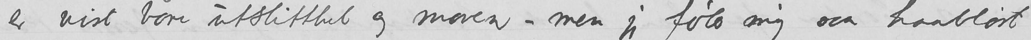er vist bare utslitthet og maven – men jeg føler mig saa haabløst
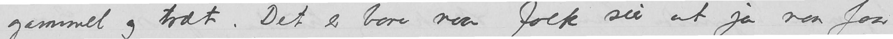gammel og træt. Det er bare naar folk sier at ja naa faar
